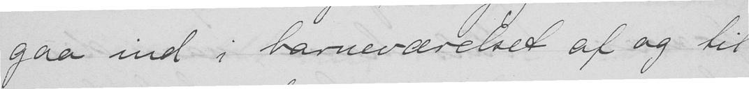gaa ind i barneværelset af og til
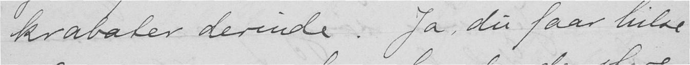krabater derinde. Ja, du faar hilse
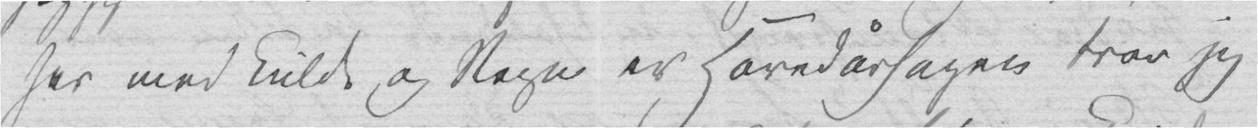her med Kulde og Regn er Hovedårsagen tror jeg
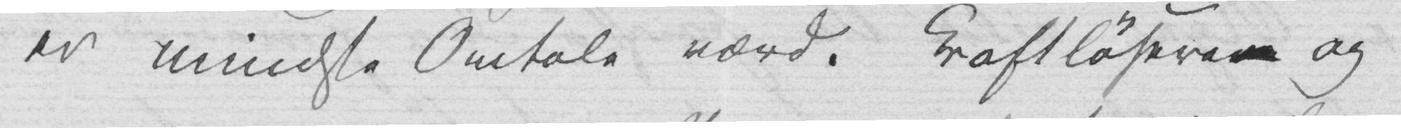er mindste Omtale værd. Kraftløsere og
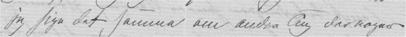jeg sige det samme om andre Ting der nager
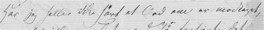Har jeg heller ikke hørt et Ord om er modtaget,
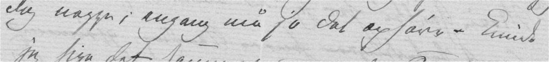dog neppe, engang må jo det ophøre – kunde
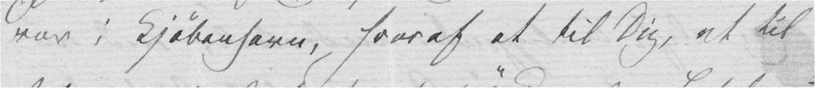var i Kjøbenhavn, hvoraf et til Dig, et til
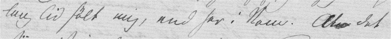lang Tid følt mig, end her i Rom. At det
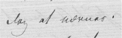dog at nævnes.
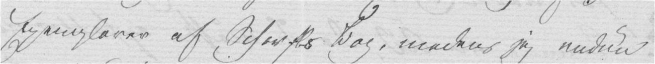Exemplarer af Schows Bog, medens jeg endnu
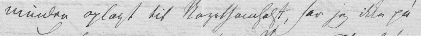mindre oplagt til Nogetsomhelst, har jeg ikke på
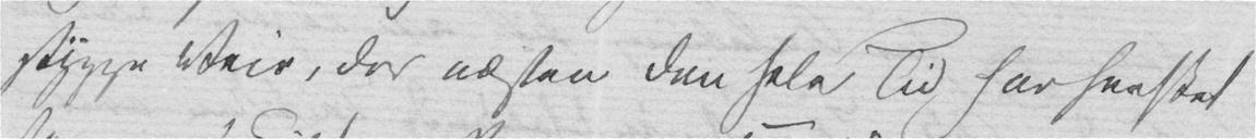stygge Veir, der næsten den hele Tid har hersket
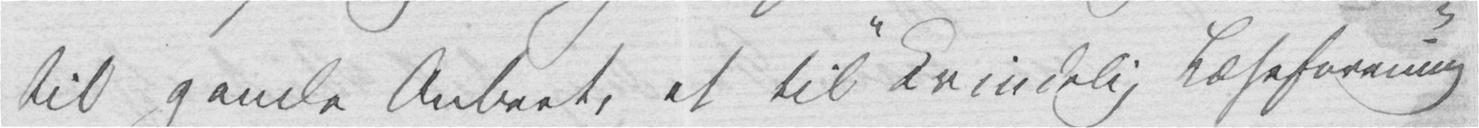til gamle Aubert, et til "Kvindelig Læseforening"
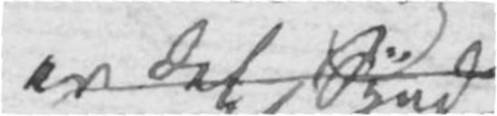er det Synd
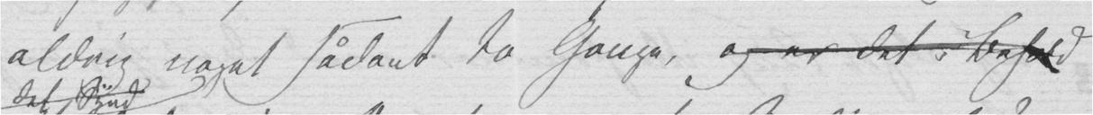aldrig noget sådant to Gange, og er det i Behold
